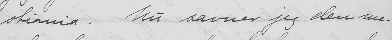stiania. Nu savner jeg den me-
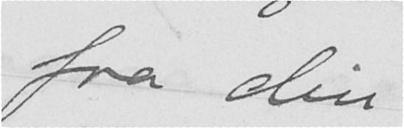fra din
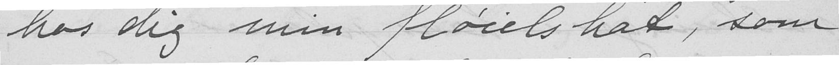hos dig min fløielshat, som
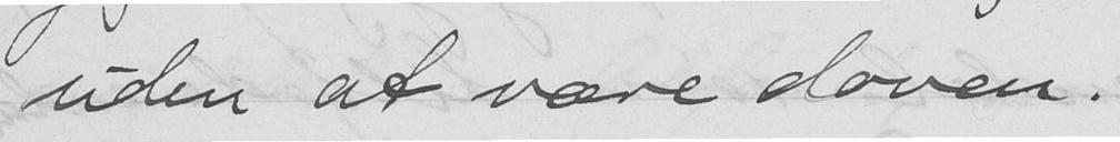uden at være doven.
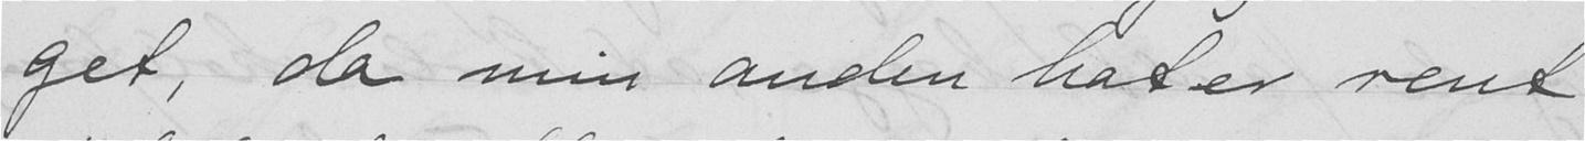get, da min anden hat er rent
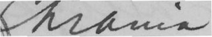Chrania
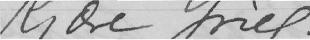Kjære Grieg!
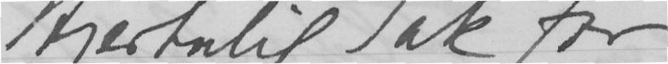Hjertelig Tak for
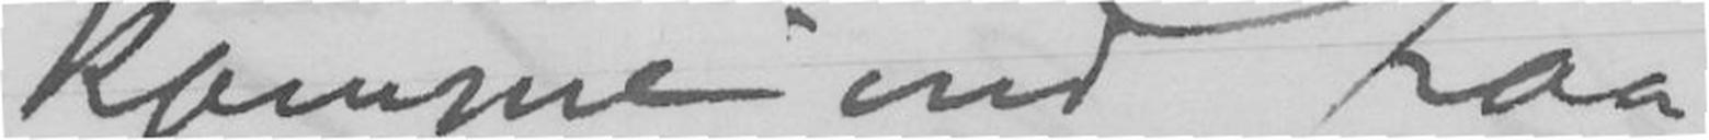komme ind paa
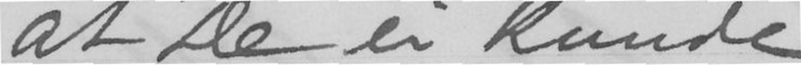at De ei kunde
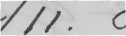12/11. 88.
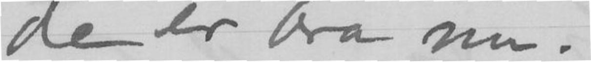de er bra nu. -
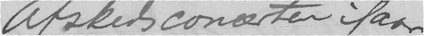Afskedconcerten igaar
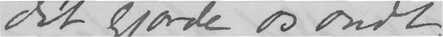det gjorde os ondt,
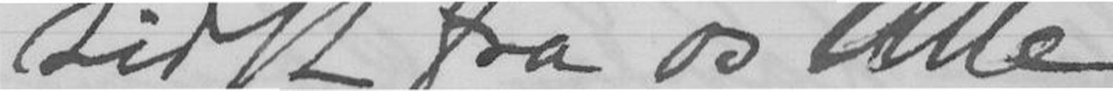sidst fra os Alle;
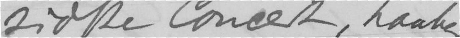sidste Concert, haaber
